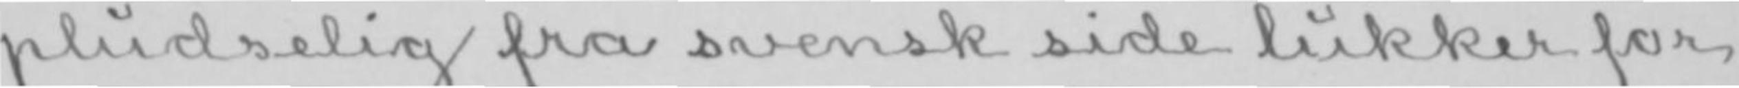pludselig fra svensk side lukker for
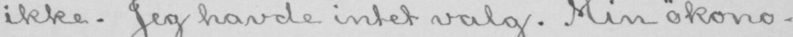ikke. Jeg havde intet valg. Min økono-
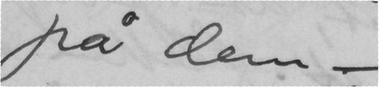på dem -
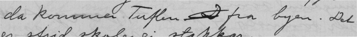da kommer Tuften  fra byen. Det
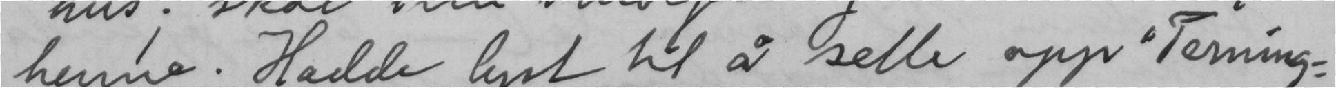henne. Hadde lyst til å sette opp. "Terning-
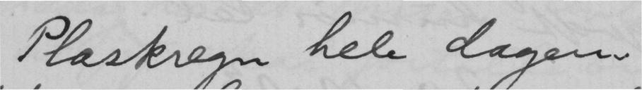Plaskregn hele dagen.
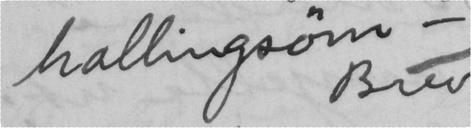hallingsøm -
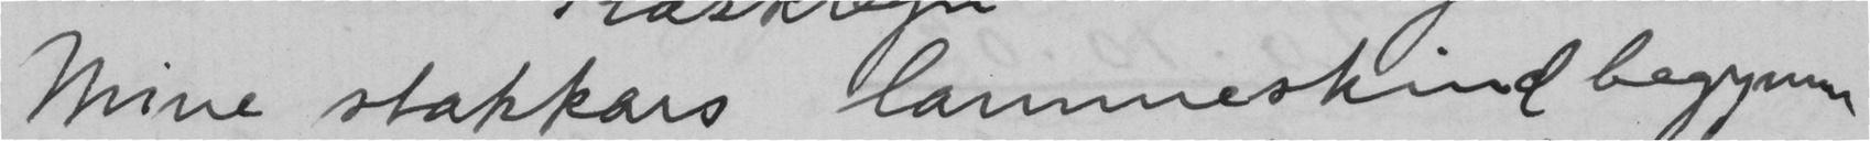Mine stakkars lammeskind begynner
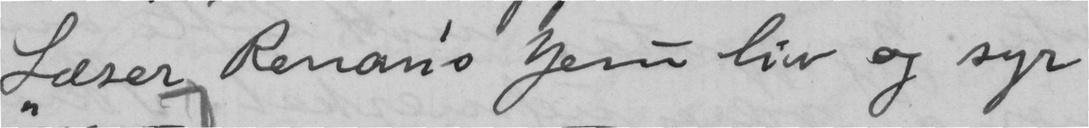Læser Renan's Jesu liv og syr
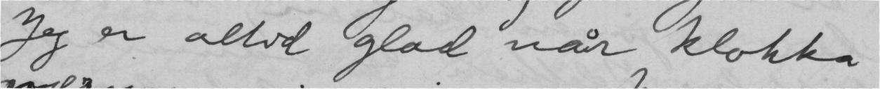Jeg er altid glad når klokka
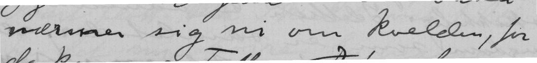mærmer sig ni om kvelden, for
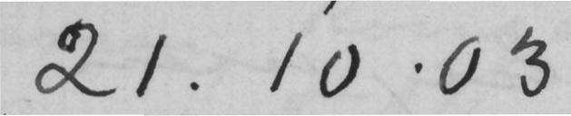21.10.03
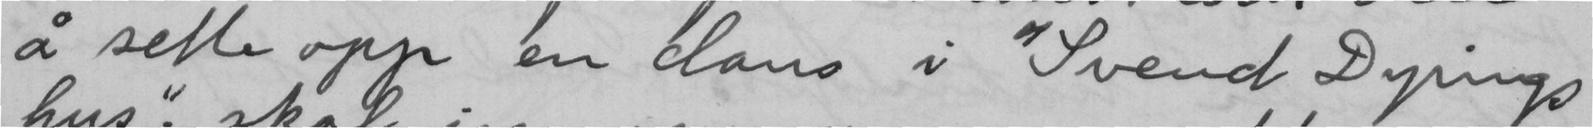å sette opp en dans i "Svend Dyrings
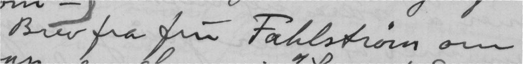Brev fra fru Fahlstrøm om
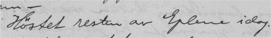Høstet resten av Eplene idag.
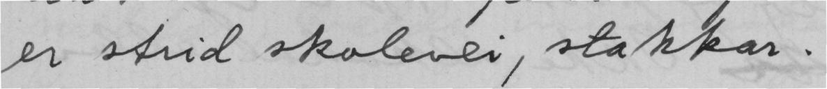er strid skolevei, stakkar.
